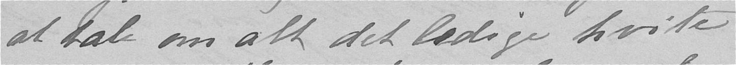at tale om alt det ledige hvite
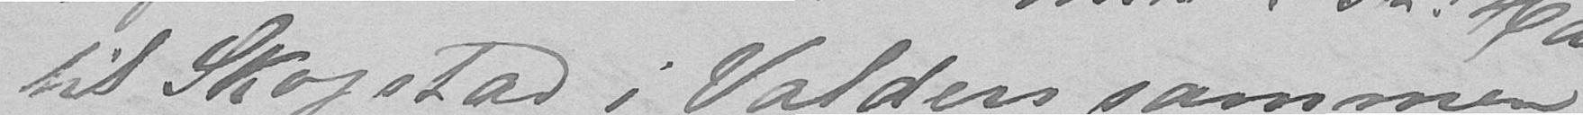til Skogstad i Valders sammen
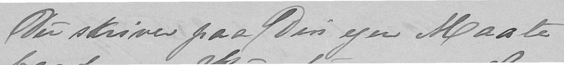Du skriver paa Din egen Maate
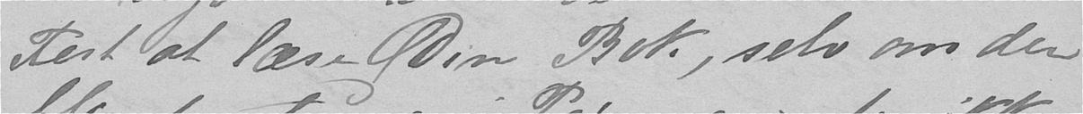Fest at læse Din Bok, selv om den
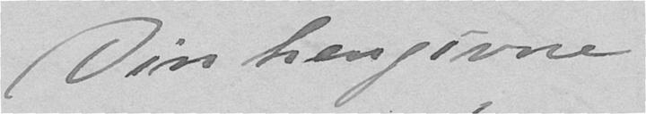Din hengivne
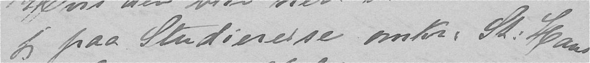jeg paa Studiereise omkr. St: Hans
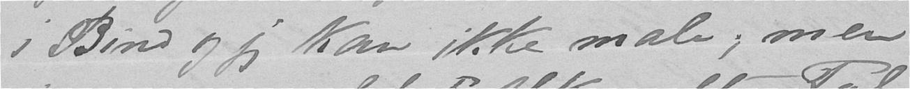i Bind og jeg kan ikke male; men
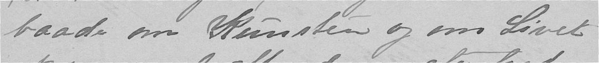baade om Kunsten og om Livet
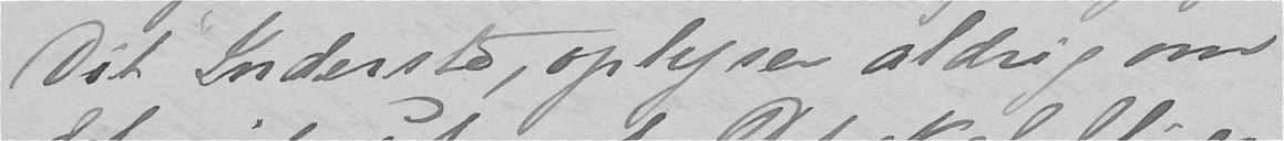Dit Inderste, oplyser aldrig om
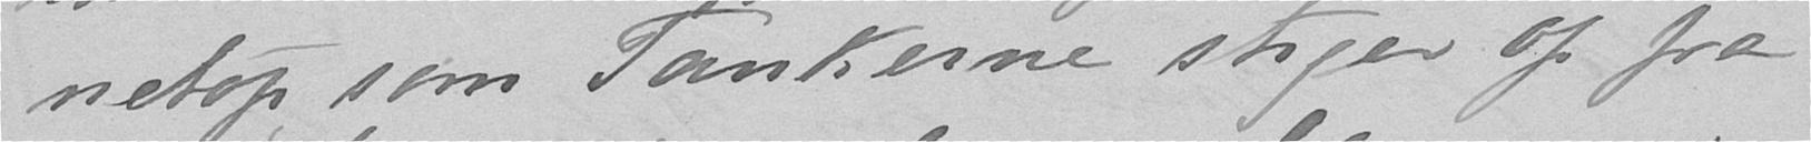netop som Tankerne stiger op fra
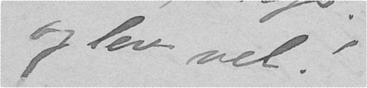og leve vel!
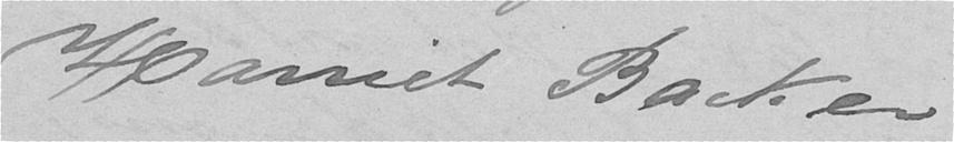Harriet Backer
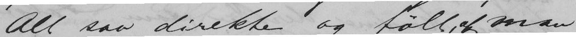Alt saa direkte og følt, at man
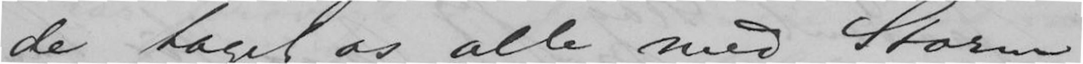de taget os alle med Storm
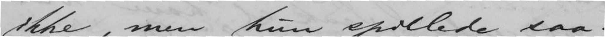ikke, men hun spillede saa-
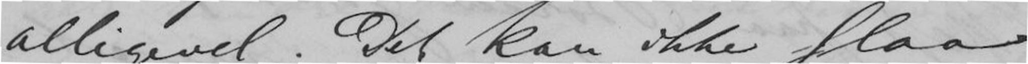alligevel. Det kan ikke slaa
